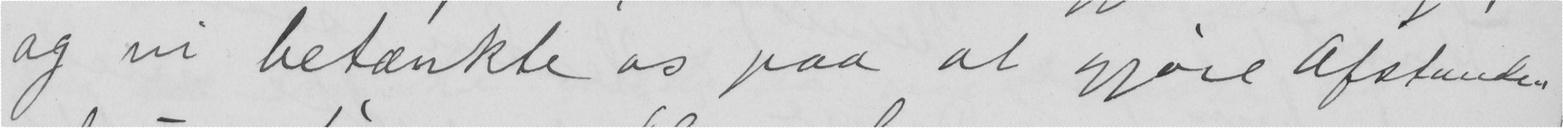og vi betænkte os paa at gjøre Afstanden
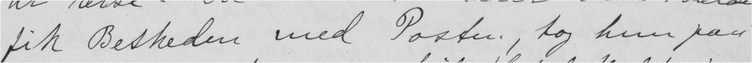fik Beskeden med Posten, tog hun paa
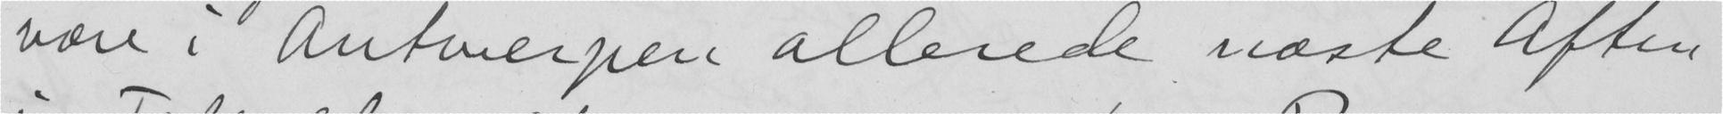være i Antwerpen allerede næste Aften
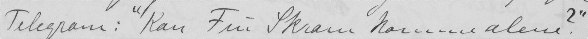Telegram: "Kan Fru Skram komme alene?"
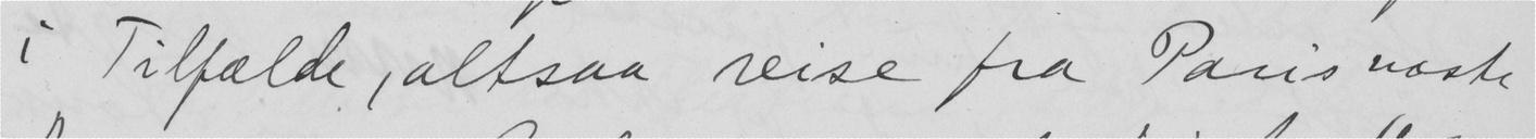i Tilfælde, altsaa reise fra Paris næste
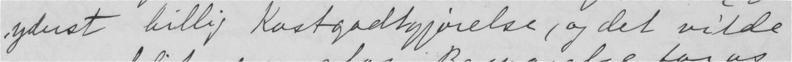yderst billig Kostgodtgjørelse, og det vilde
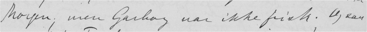Morgen; men Garbor var ikke frisk. Og saa
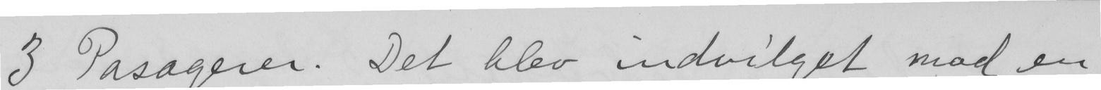3 Pasagerer. Det blev indvilget mod en
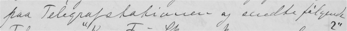paa Telegrafstationen og snedte følgende
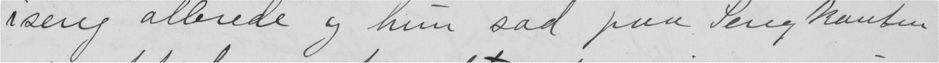iseng allerede og hun sad paa Sengkanten
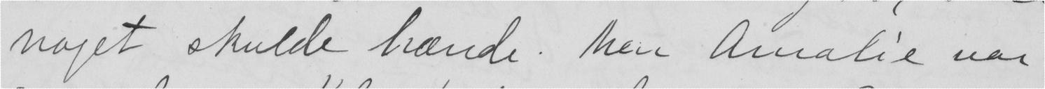noget skulde hænde. Men Amalie var
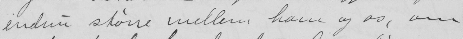endnu større mellem ham og os, om
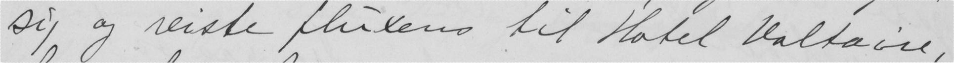sig og reiste fluxens til Hotel Voltaire,
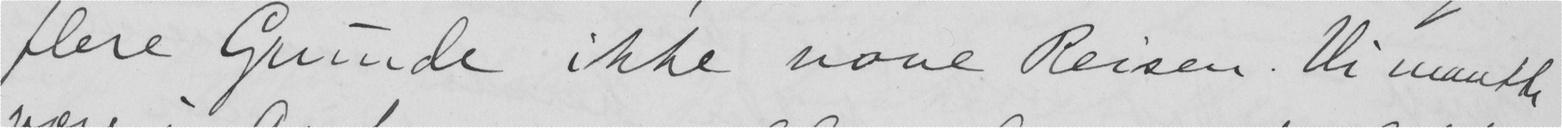flere Grunde ikke vove Reisen. Vi maatte
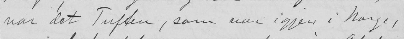var det Tuften, som var igjen i Norge,
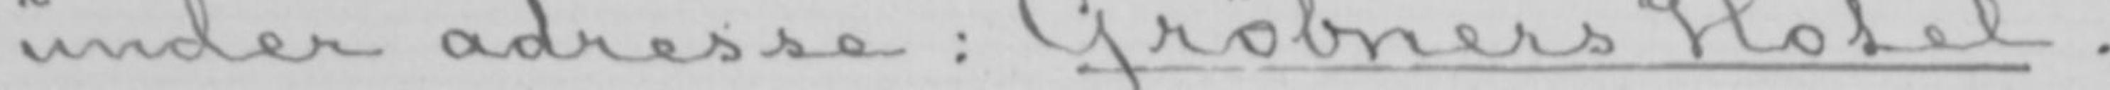under adresse: Gröbners Hotel.
